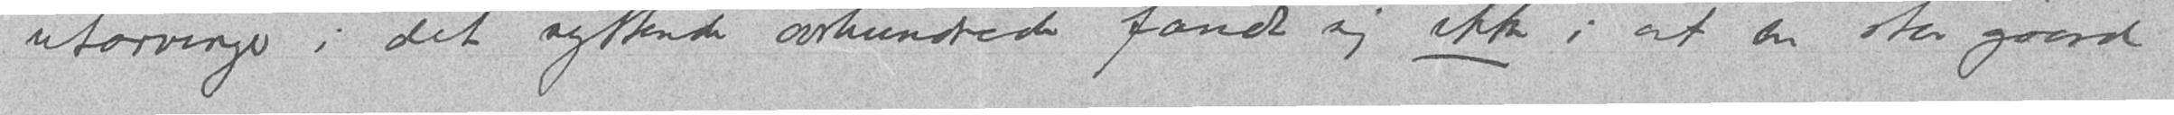utarvinger i det syttende aarhundrede fandt sig ikke i at en stor gaard
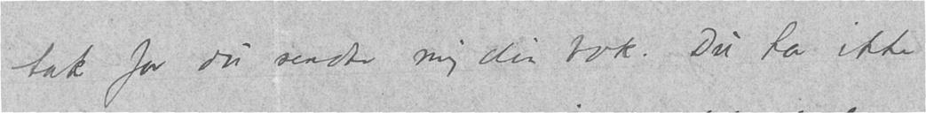tak for du sendte mig din bok. Du har ikke
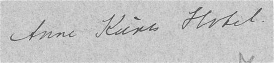Anne Kures Hotel.
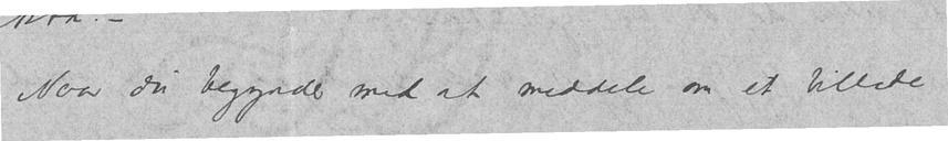Naar du begynder med at meddele om et billede
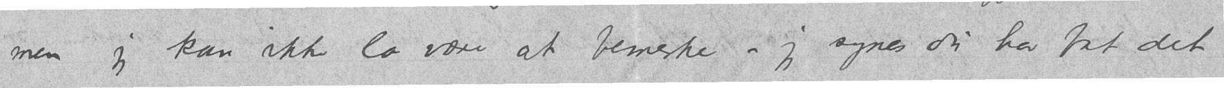men jeg kan ikke la være at bemerke – jeg synes du har tat det
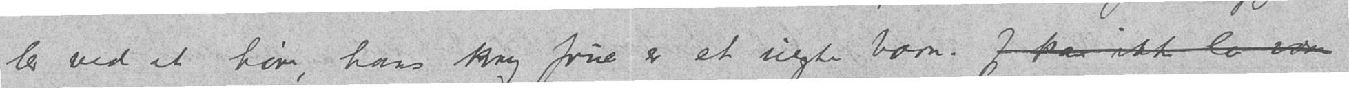ler ved at høre, hans kry frue er et uegte barn. Jeg kan ikke la være
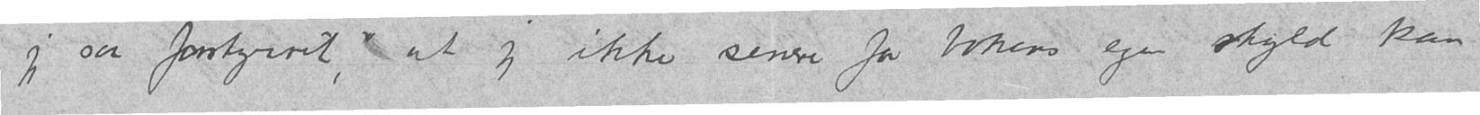jeg saa forstyrret, at jeg ikke senere for bokens egen skyld kan
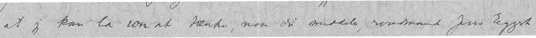at jeg kan la være at tænke, naar du meddeler, raadmand Jens Eggert
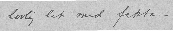lovlig let med fakta. -
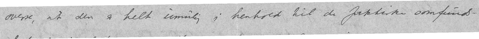overse, at den er helt umulig i henhold til de faktiske samfunds-
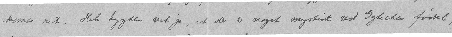kones ret. Hele bygden vet jo, at der er noget mystisk ved Gylickes fødsel,
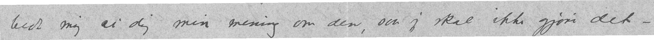bedt mig si dig min mening om den, saa jeg skal ikke gjøre det –
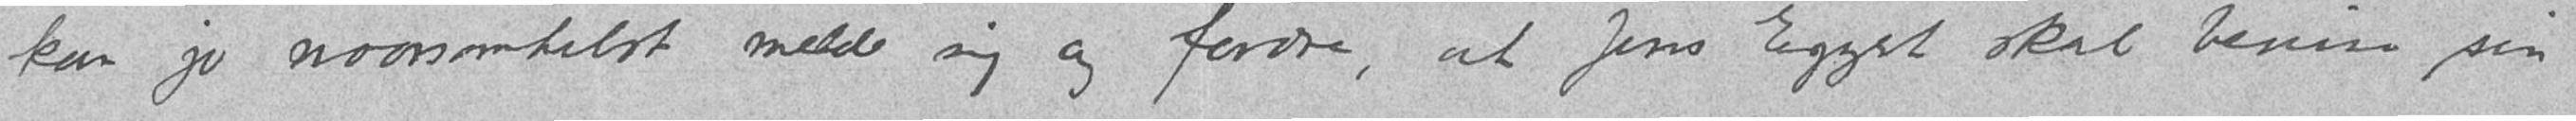kan jo naarsomhelst melde sig og fordre, at Jens Eggert skal bevise sin
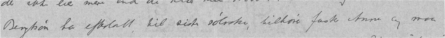Bengtsøn har efterlatt, til siste sølvske, tilhører faster Anne og maa
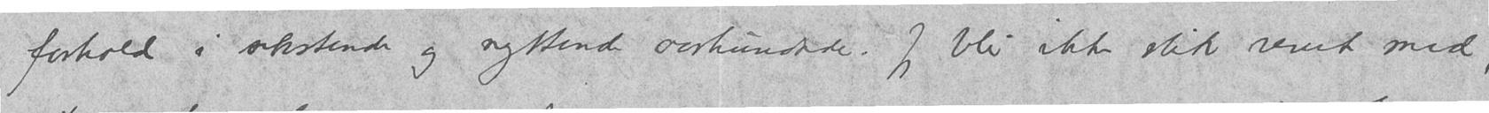forhold i sekstende og syttende aarhundrede. Jeg blir ikke slik revet med,
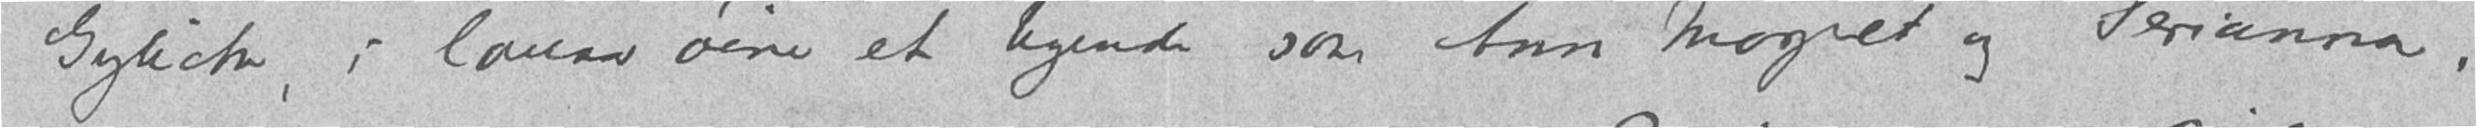Gylicke, i lovens øine et tyende som Ann Magret og Serianna,
